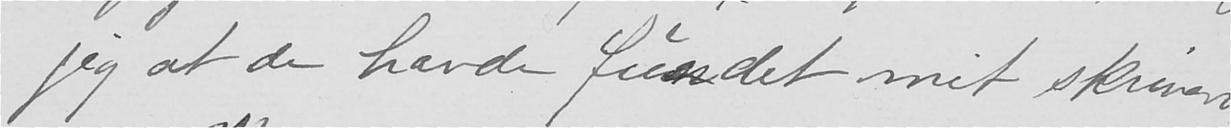jeg at de havde fundet mit skriveri
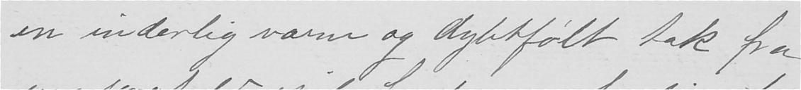en inderlig varm og dybtfølt tak fra
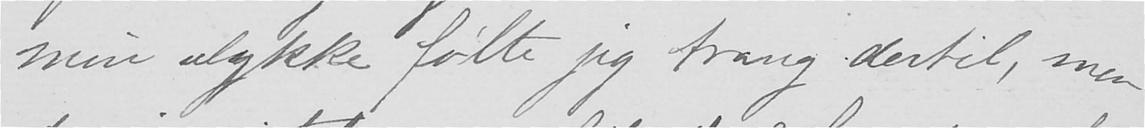min ulykke følte jeg trang dertil, men
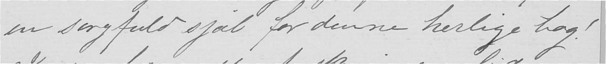en sorgfuld sjæl for denne herlige bog!
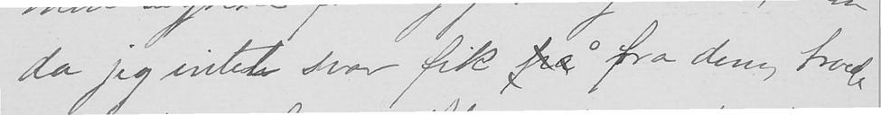da jeg intet svar fik på fra dem, trods
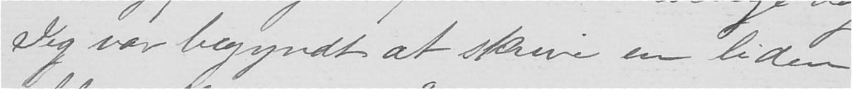Jeg var begyndt at skrive en liden
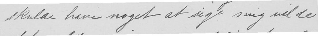skulde have noget at sige mig vilde
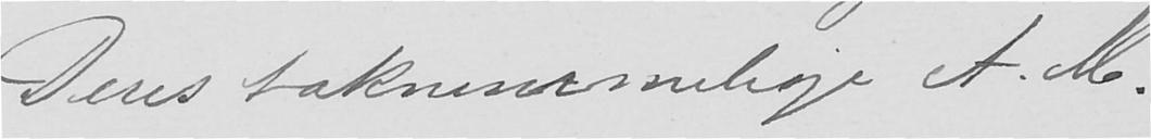Deres taknemmelige A.M.
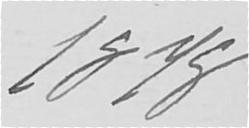1878
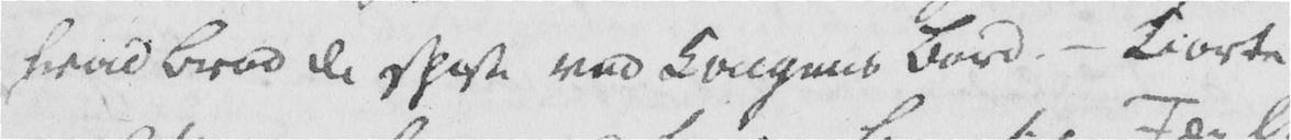hvad Brød de spiste ved Kongens Bord. - Kiørte
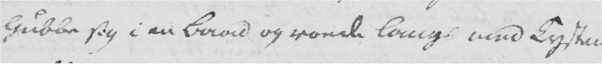Gubbe sig i en Baad og roede langs med Kysten
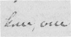ken, om
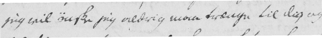jeg vil ønske jeg aldrig maa trænge til dig og
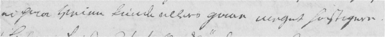ei paa Veien kunde ellers gaae meget hastigere.
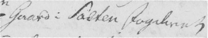Gaard i Salten Fogderiet
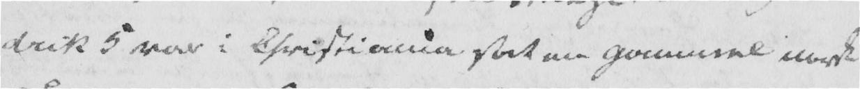derik 5 var i Christiana sat en gammel norsk
